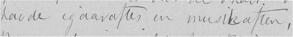havde igaaraftes en musikaften,
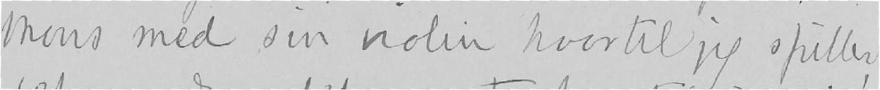Mons med sin violin hvortil jeg spiller,
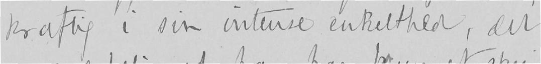kraftig i sin intense enkelthed, det
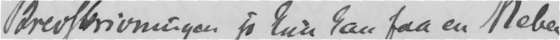Brevskrivningen jo kun kan faa en Neben-
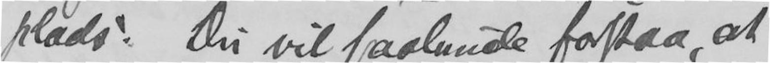plads! Du vil saalunde forstaa, at
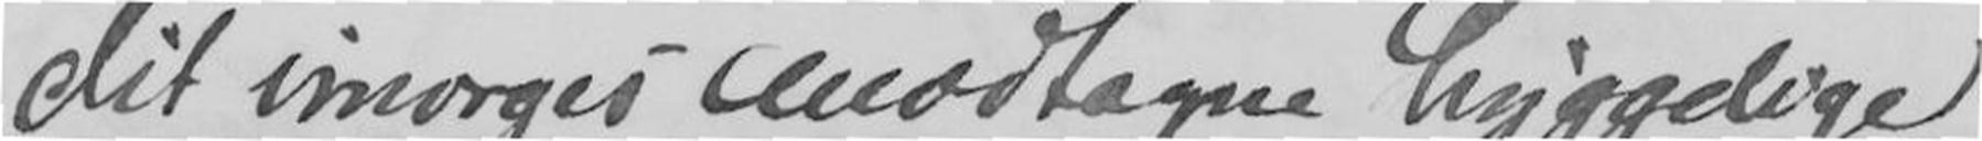dit imorges modtagne hyggelige
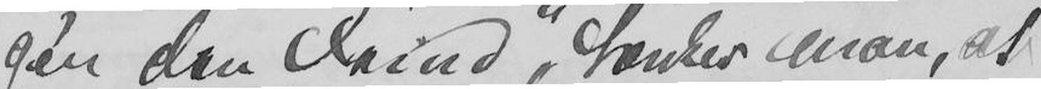gen den Faind", tænker man, at
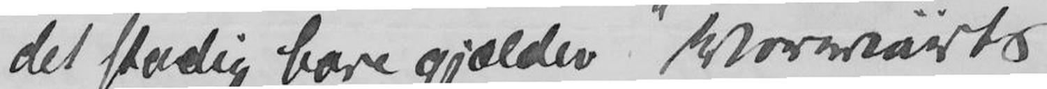det stadig bare gjælder "Vorvarts
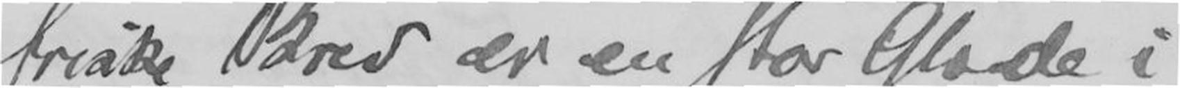friske Brev er en stor Glæde i
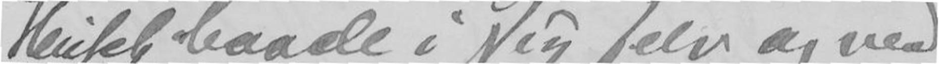Huset baade i sig selv og ved
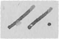11.
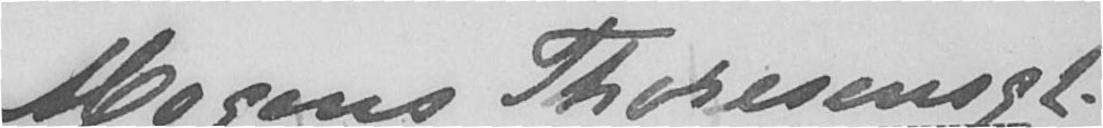Mogens Thoresensgt.
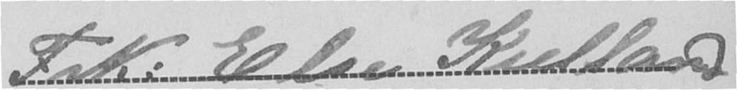Frk. Else Kielland
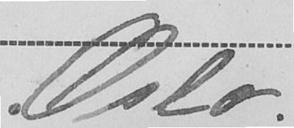Oslo.
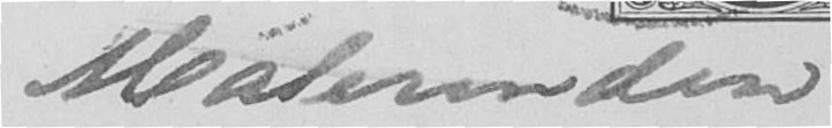Malerinden
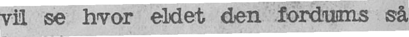vil se hvor edelt den fordums så
